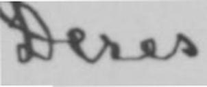Deres
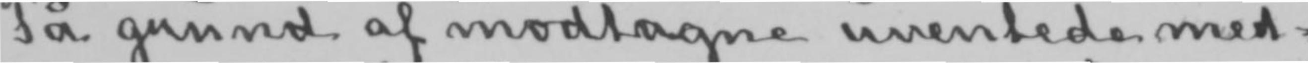På grund af modtagne uventede med-
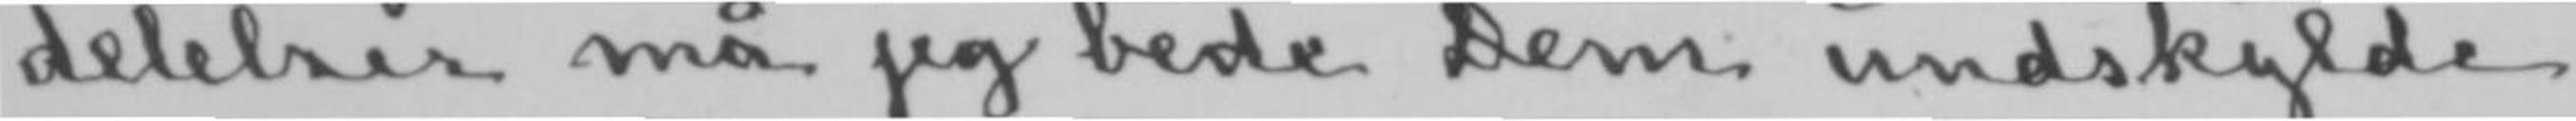delelser må jeg bede Dem undskylde
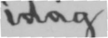idag.
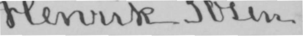Henrik Ibsen.
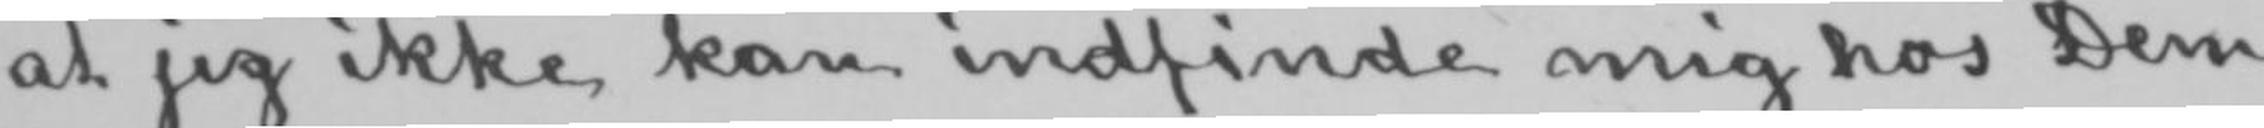at jeg ikke kan indfinde mig hos Dem
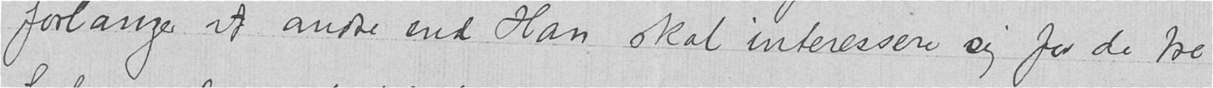forlanger at andre end Han skal interessere sig for de tre
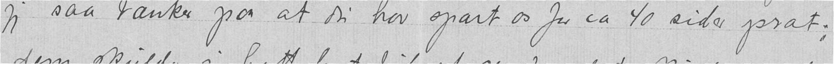jeg saa tænker paa at du har spart os for ca 40 sider prat;
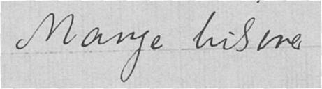Mange hilsener
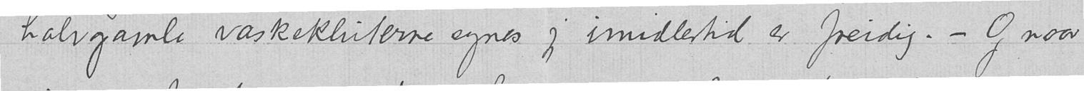halvgamle vaskekluterne synes jeg imidlertid er freidig. - Og naar
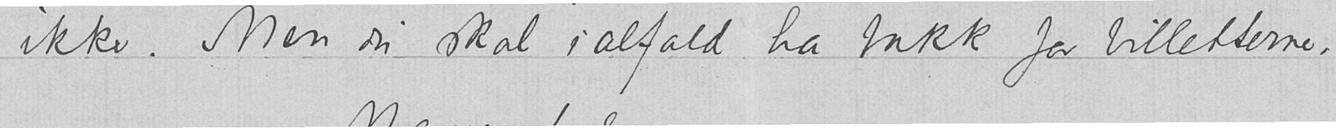ikke. Men du skal ialfald ha takk for billetterne.
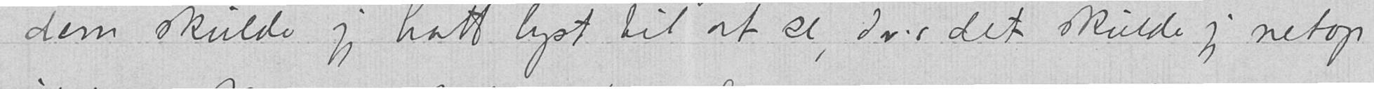dem skulde jeg hatt lyst til at se, dv.s det skulde jeg netop
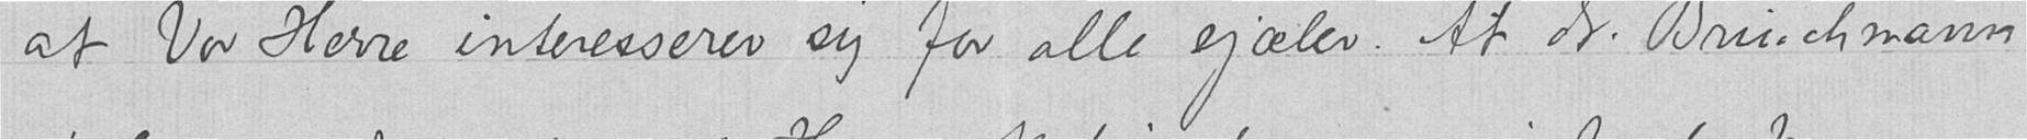at Vor Herre interesserer sig for alle sjæler. At dr. Brinchmann
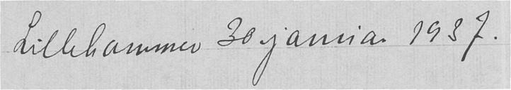Lillehammer 30. januar 1937.
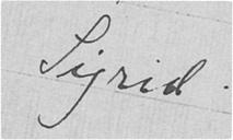Sigrid
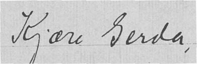Kjære Gerda,
